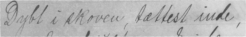Dybt i skoven, tættest inde,
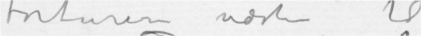torturere næsten til
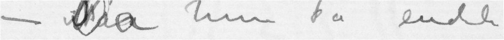– Da hun da endeli
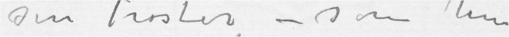sin Trøster – som hun
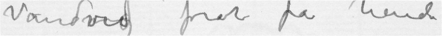Vandvid forat få hende
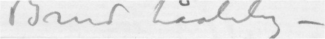Brud tåalelig –
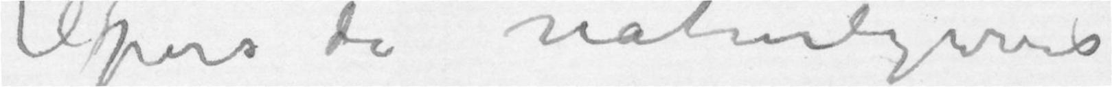tilpers da naturligvis
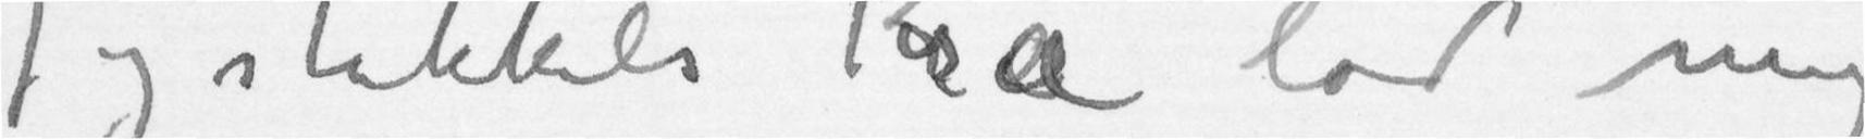Jeg stakkels Kræ lod mig
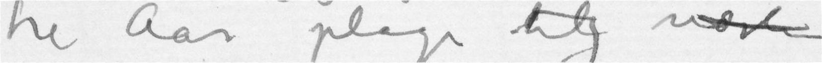tre Aar plage tilog næste
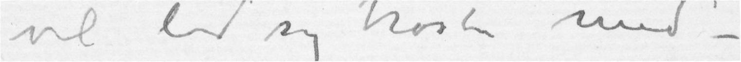vel lod sig trøste med –
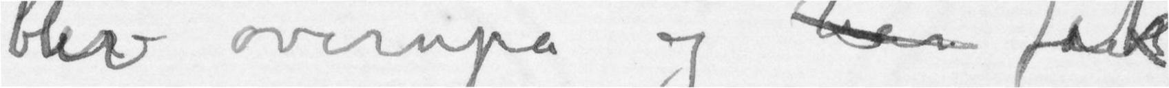blev ovenpå og har fik
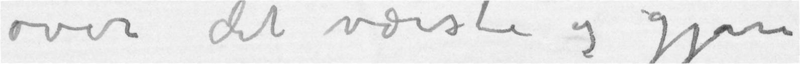over det værste og gjøre
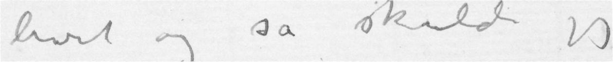levet og så skulde jeg
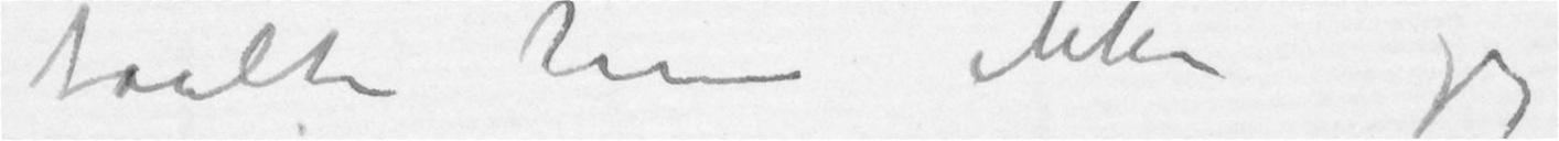taalte hun ikke jeg
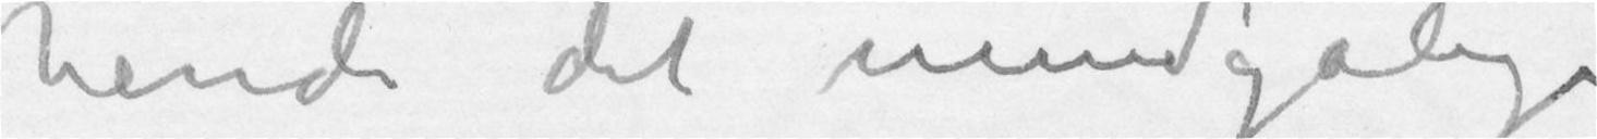hende det uundgåelige
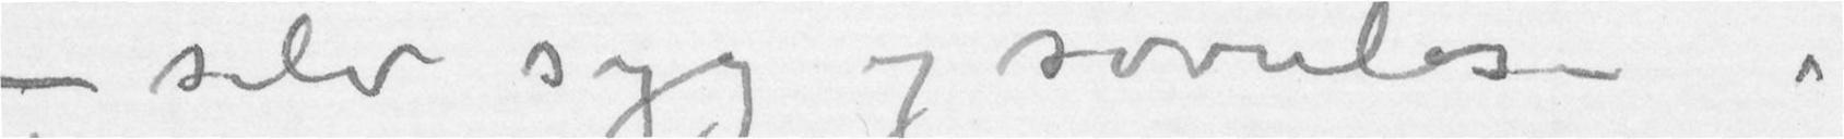– selv syg og søvnløs – i
